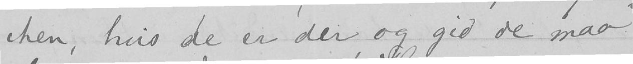chen, hvis de er der, og gid de maa
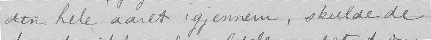den hele aaret igjennem, skulde de
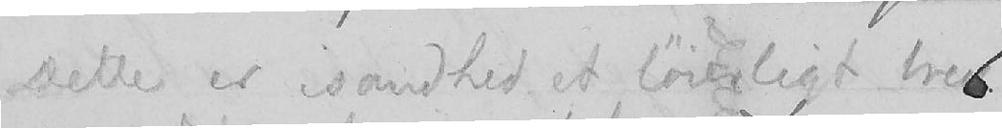Dette er isandhed et løierligt brev,
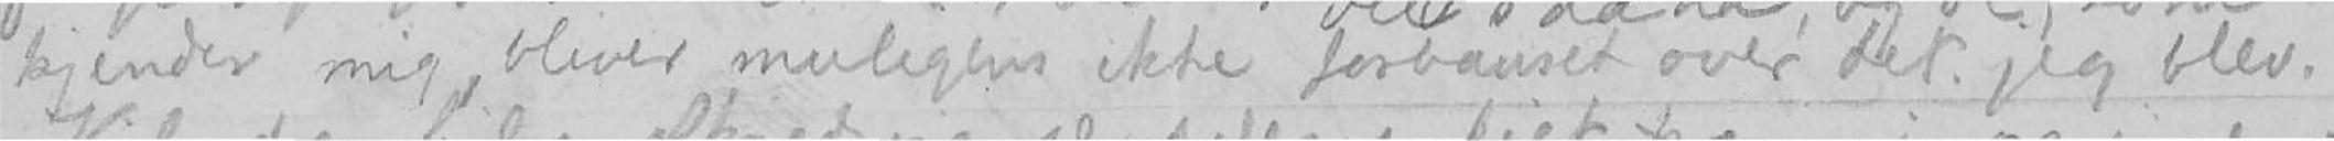kjender mig, bliver muligens ikke forbauset over det. jeg blev.
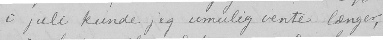i juli kunde jeg umulig vente længer,
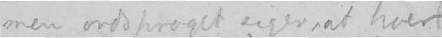men ordsproget siger, at hver
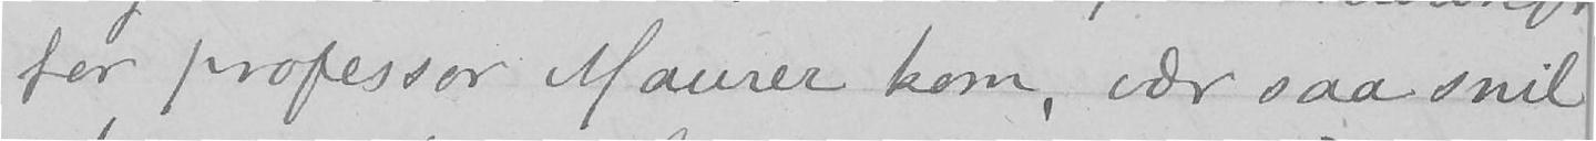før professor Maurer kom, vær saa snil
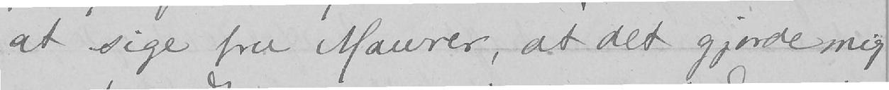at sige fru Maurer, at det gjorde mig
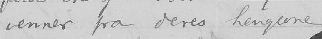venner fra deres hengivne
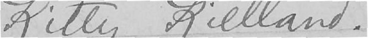Kitty Kielland
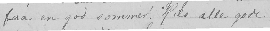faa en god sommer. Hils alle gode
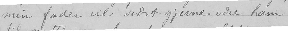min fader vil svært gjerne være ham
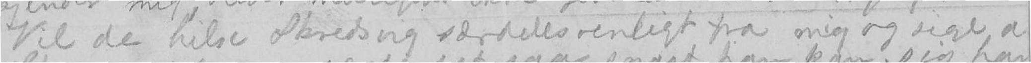Vil de hilse Skredsvig særdeles venligt fra mig og sige at
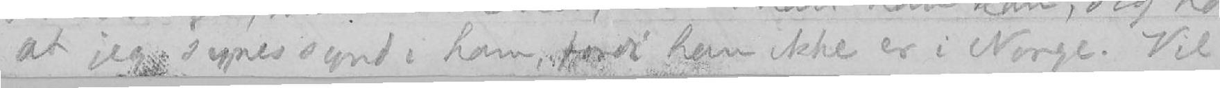at jeg synes synd i ham, fordi han ikke er i Norge. Vil
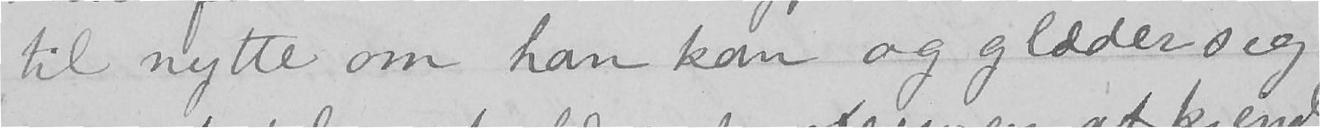til nytte om han kan og glæder sig
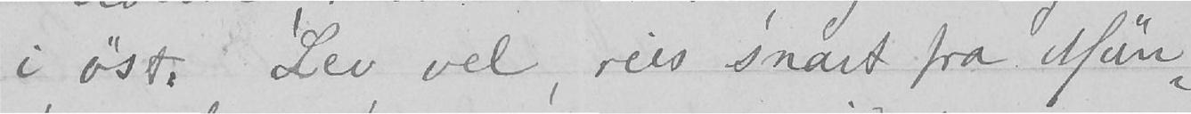i øst. Lev vel, reis snart fra Mün-
